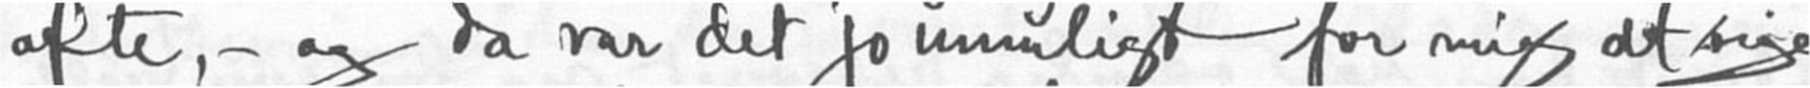ofte, - og da var det jo umuligt for mig at sige
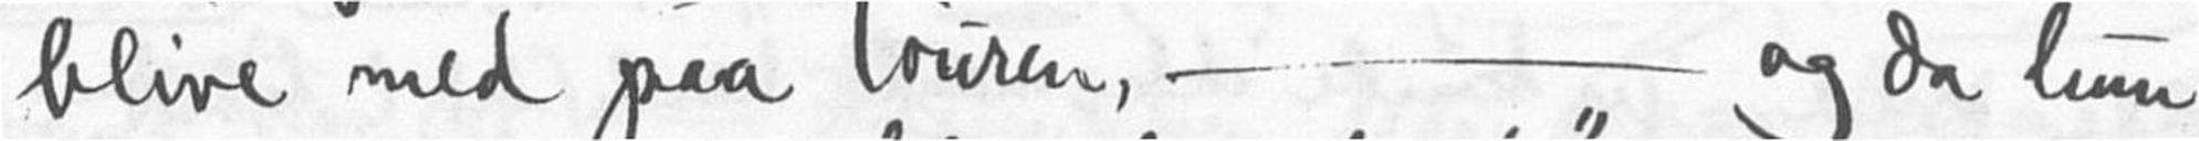blive med paa touren, - og da hun
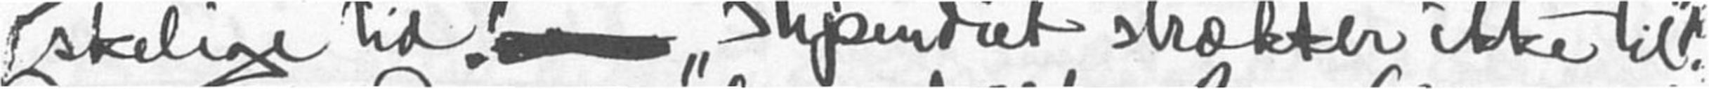skelige tid"! - "stipendiet strækker ikke til".
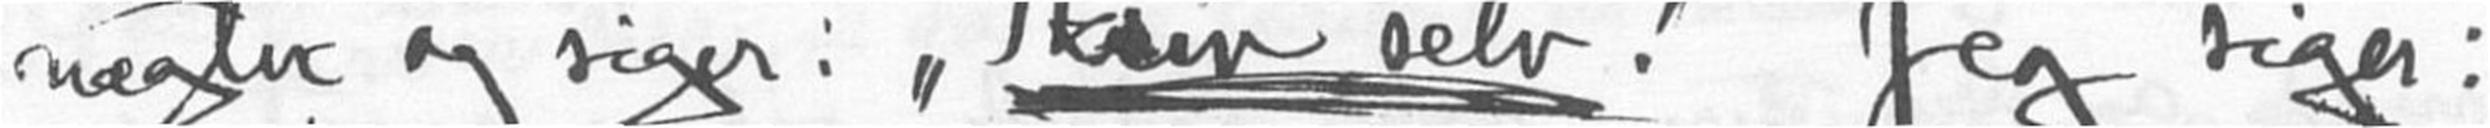nægter og siger : "Skriv selv!" Jeg siger:
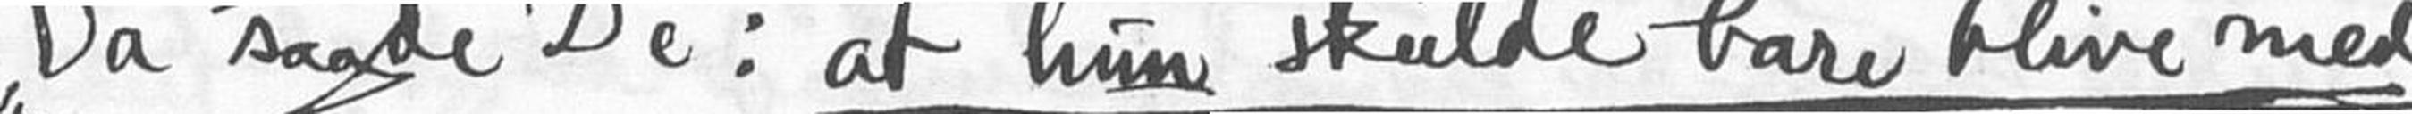Da sagde De : at hun skulde bare blive med.
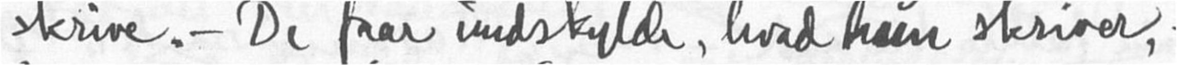skrive, - De faar undskylde, hvad hun skriver, -
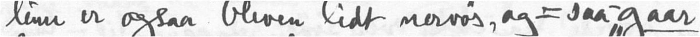hun er ogsaa bleven lidt nervøs, og saa gaar
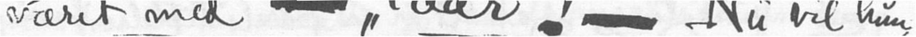været med "iaar"! - Nu vil hun,
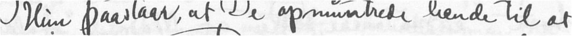Hun paastaar, at De opmuntrede hende til at
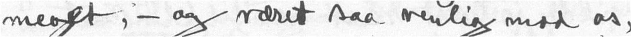meget, - og været saa venlig mod os,
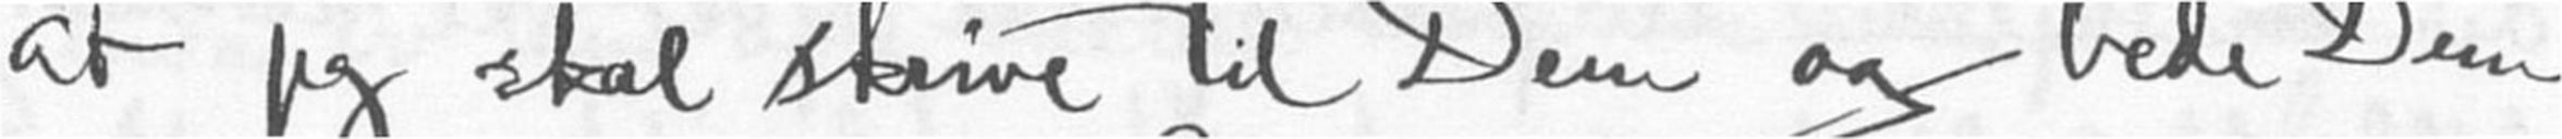at jeg skal skrive til Dem og bede Dem
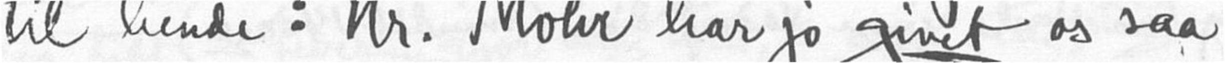til hende: Hr. Mohr har jo givet os saa
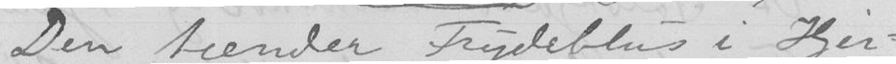Den tænder Frydeblus i Hjer-
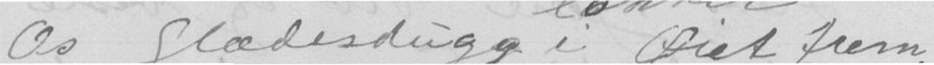Os Glædesdugg i Øiet frem.
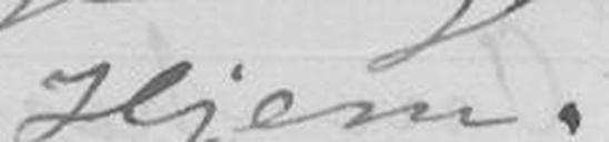Hjem.
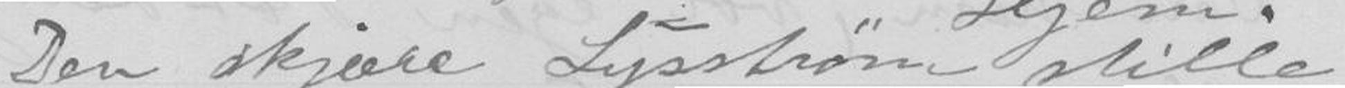Den skjære Lysstrøm stille
